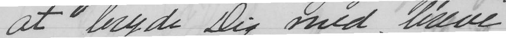at bryde Dig med breve
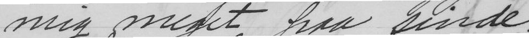mig meget paa sinde
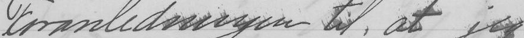Foranledningen til, at jeg
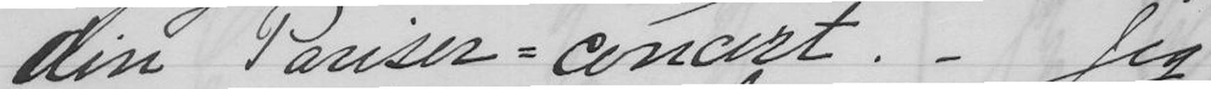din Pariser=concert. - Jeg
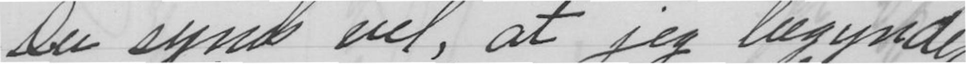Du synes vel, at jeg begynder
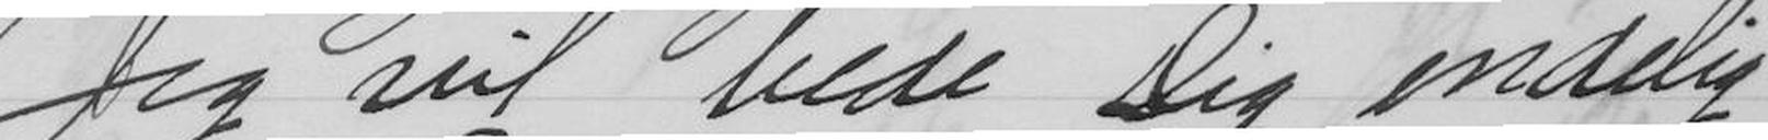Jeg vil bede Dig endelig
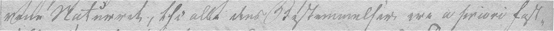rene Naturret, thi alle dens Bestemmelser ere a priori fast-
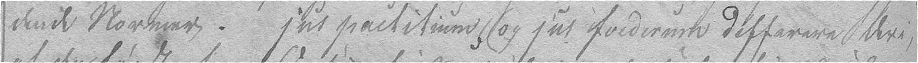dende Normer. jus pactitum og jus foedorum differere deri,
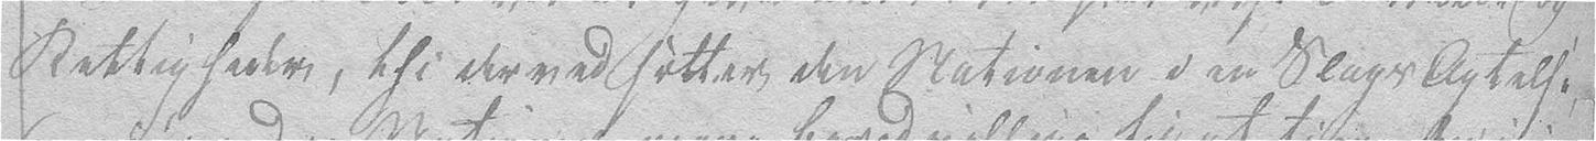Rettigheder, thi derved sætter den Nationen i en Slags Agtelse,
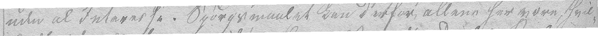uden al Interesse. Spørgsmaalet kan derfor allene her være hvil-
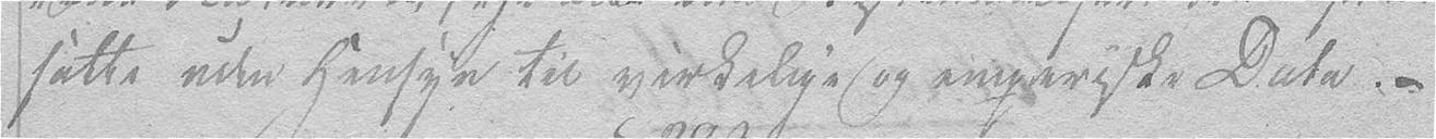satte uden Hensyn til virkelige og empiske Data. –
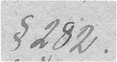§ 282.
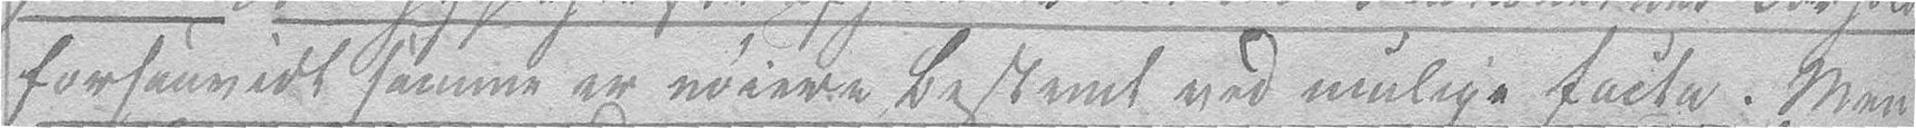forsaavidt samme er nøiere bestemt ved mulige facta. Men
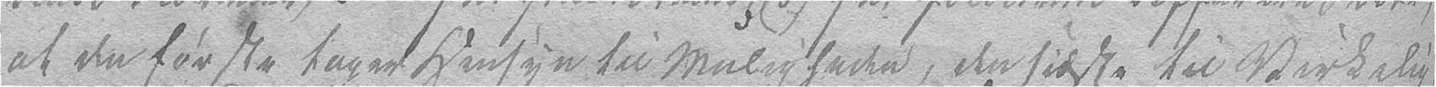at den første tager Hensyn til Muligheden, den sidste til Virkelig-
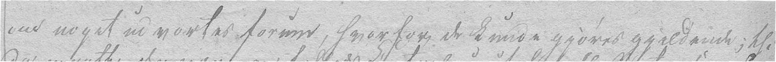om noget udvortes Forum, hvorfor de kunde gjøres gjeldende; thi
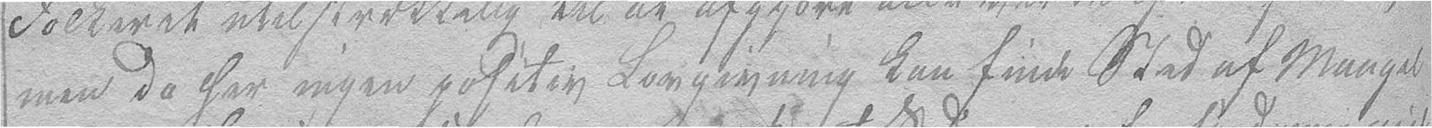men da her ingen positiv Lovgivning kan finde Sted af Mangel
